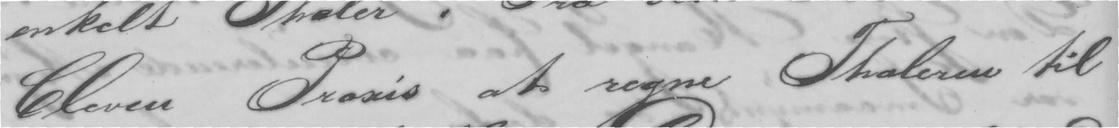bleven Praxis at regne Thaleren til
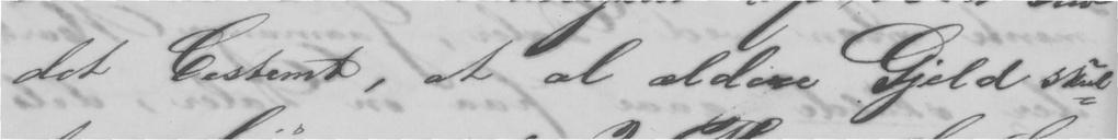det bestemt, at at ældere Gjeld skul-
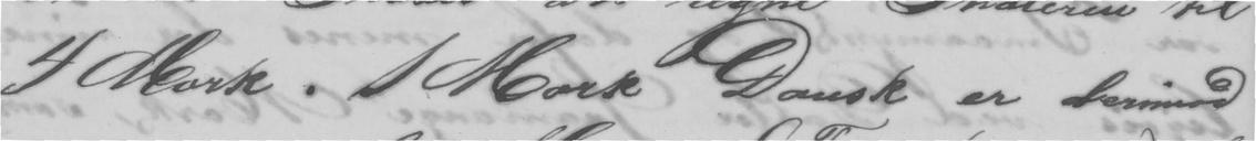4 Mark. 1 Mark Dansk er derimod
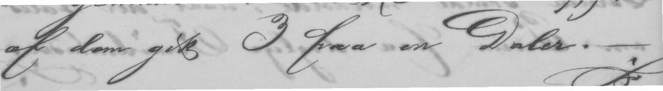af dem gik 3 paa en Daler. -
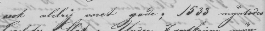nok aldrig været gode; i 1533 myntedes
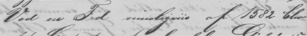Ved en Frd rimeligvis af 1582 blev
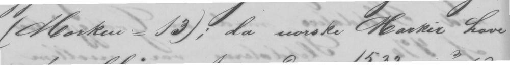(Marken = 13); da norske Marker have
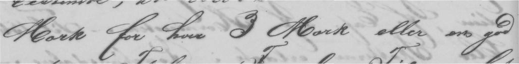Mark for hver 3 Mark eller en god
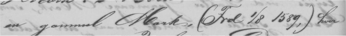en gammel Mark. (Frd 1/8 1589,) hvor
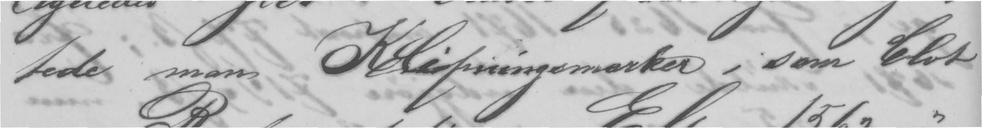tede man Klipningsmarker, som blot
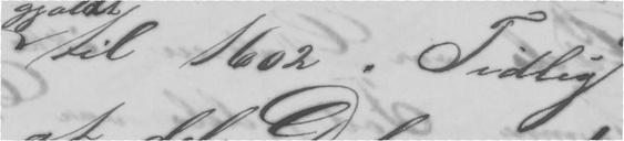til 1602. Tidlig
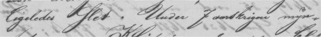ligeledes slet. Under 7 aarskrigen myn-
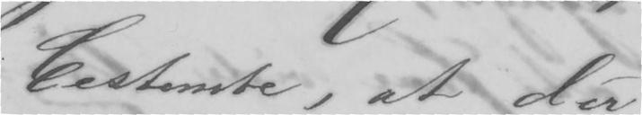bestemte, at der
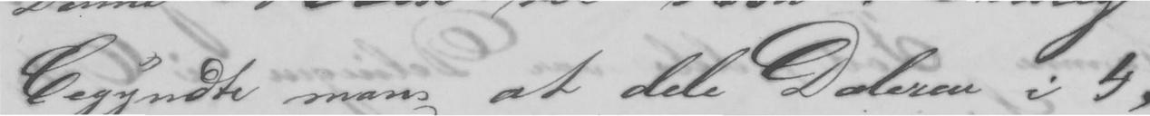begyndte man at dele Daleren i 4,
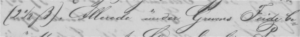(2½ ẞ). Allerede under Grevens Feide be-
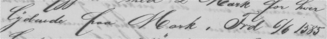lydende paa Mark . Frd 6/6 1583
